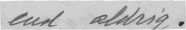end aldrig!
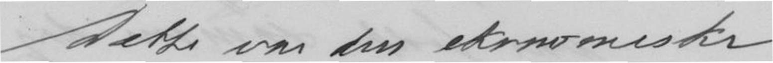Dette var den økonomiske
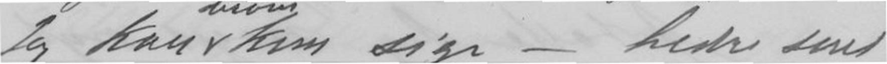Jeg kan kun sige - bedre sent
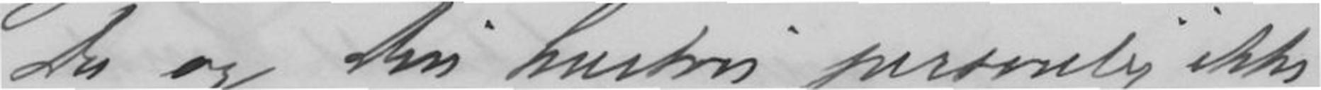Du og Din hustru personlig ikke
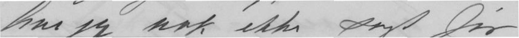har jeg nok ikke sagt før.
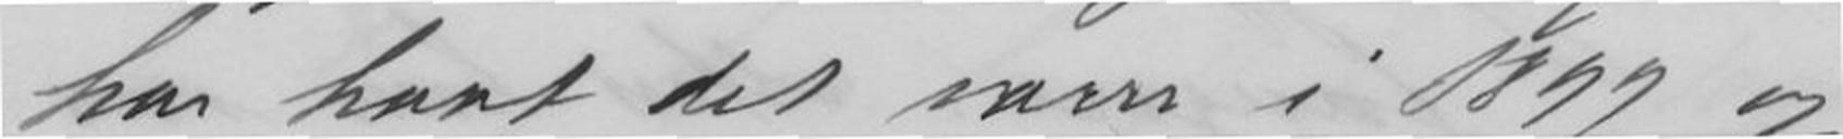har havt det værre i 1899 og
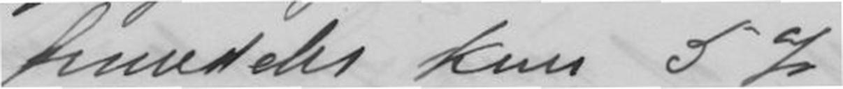fremdeles kun 5%.
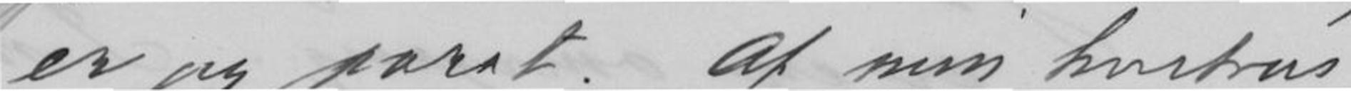er jeg parat. Af min hustrus
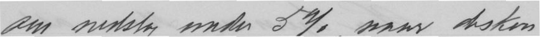om nedslag under 5%, naar diskon-
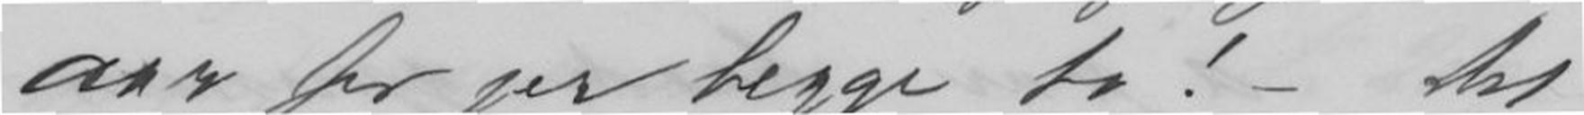aar for jer begge to! - Det
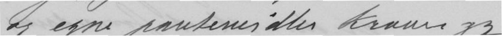og egne pantemidler kræver jeg
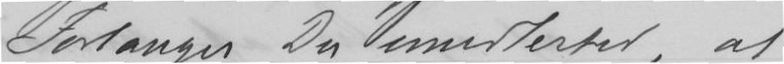Forlanger Du imidlertid, at
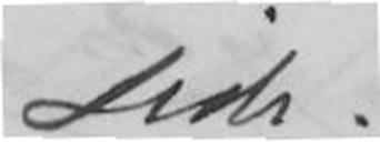Side.
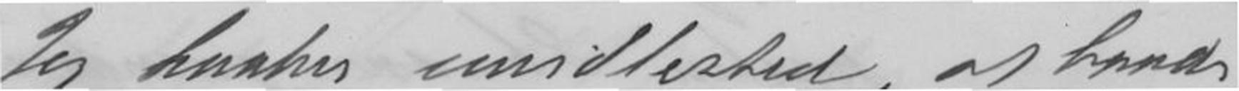Jeg haaber imidlertid, at baade
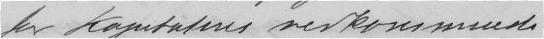for Kapitalens vedkommende.
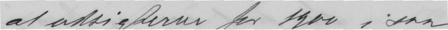at udsigterne for 1900 i saa
